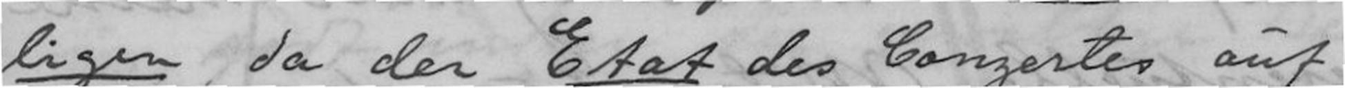-ligen, da der Etat des Conzertes auf
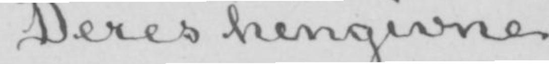Deres hengivne
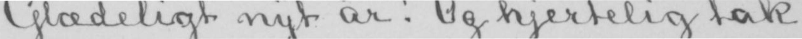Glædeligt nyt år! Og hjertelig tak
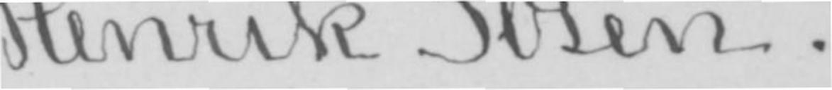Henrik Ibsen.
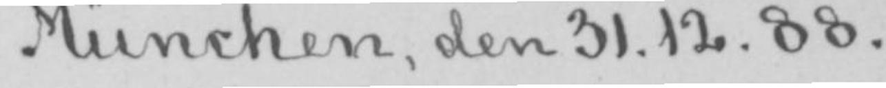München, den 31.12.88.
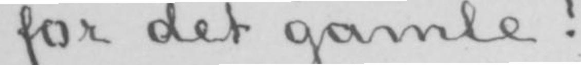for det gamle!
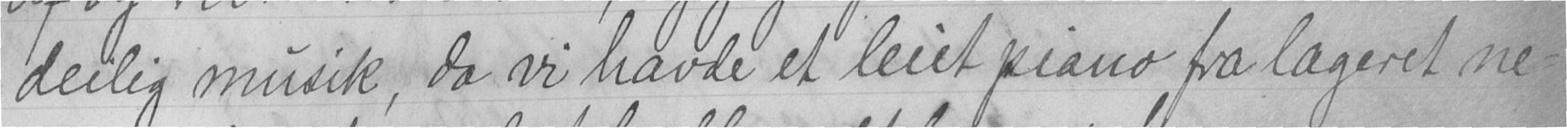deilig musik, da vi havde et leiet piano fra lageret ne-
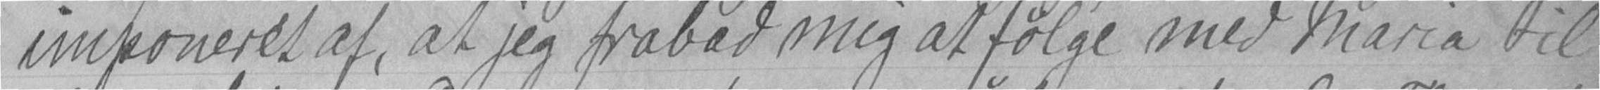imponeret af, at jeg frabad mig at følge med Maria til
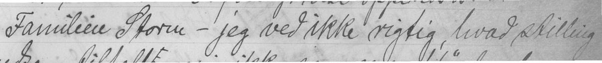Familien Storm - jeg ved ikke rigtig, hvad stilling
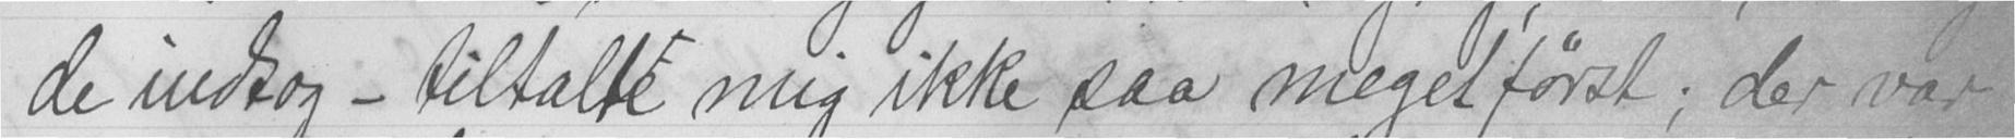de indtog - tiltalte mig ikke saa meget først; der var
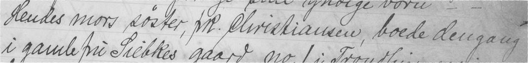Hendes mors søster, frk. Christiansen, boede dengang
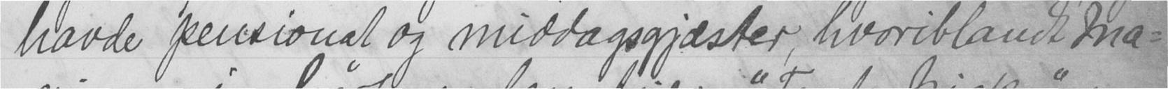havde pensionat og middagsgjæster, hvoriblandt Ma-
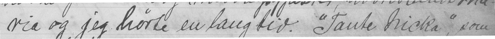ria og jeg hørte en lang tid. "Tante Nicka," som
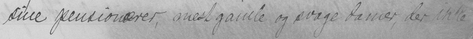sine pensionærer, mest gamle og svage damer, der ikke
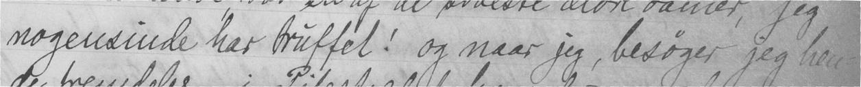nogensinde har truffet! og naar jeg, besøger jeg hen-
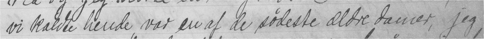vi kaldte hende, var en af de sødeste ældre damer, jeg
